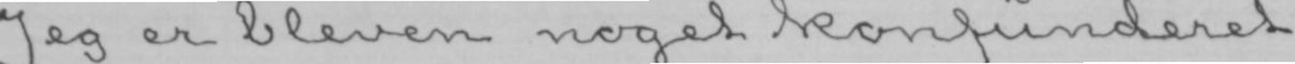Jeg er bleven noget konfunderet
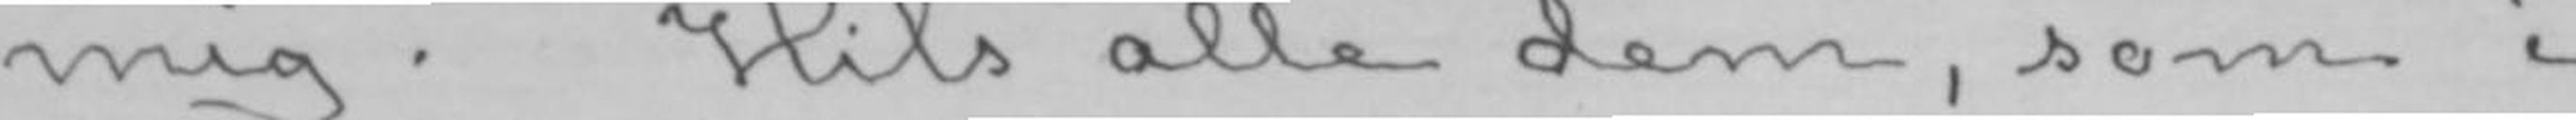mig. Hils alle dem, som i
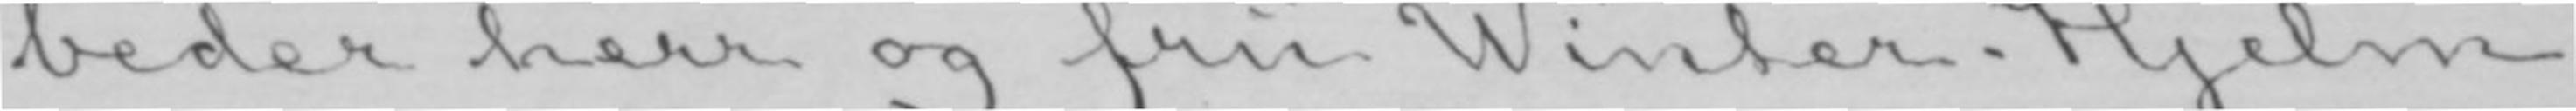beder herr og fru Winter-Hjelm
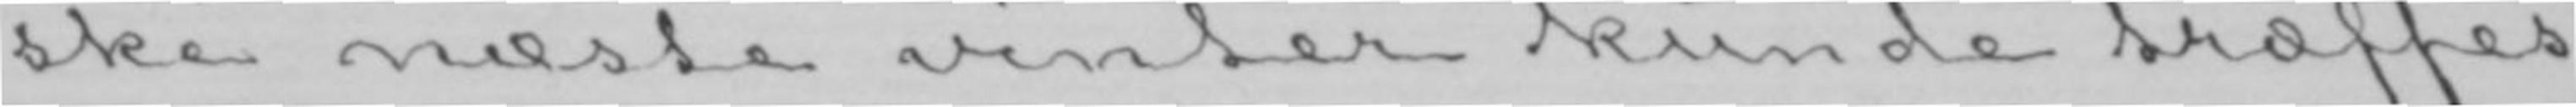ské næste vinter kunde træffes
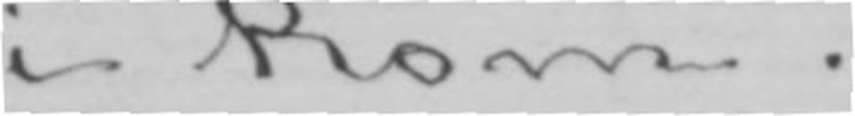i Rom.
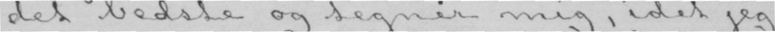det bedste og tegner mig, idet jeg
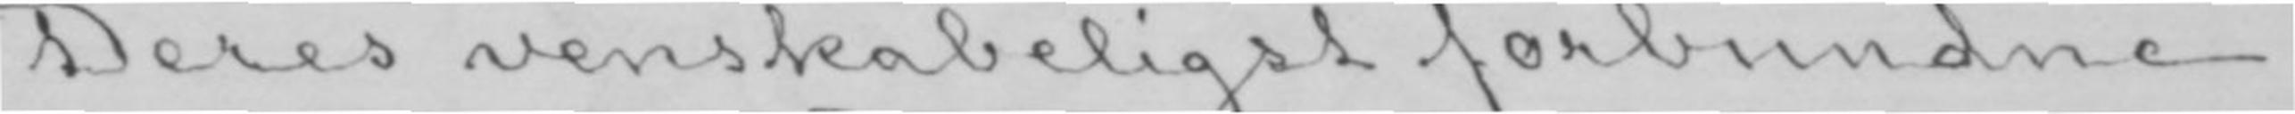Deres venskabeligst forbundne
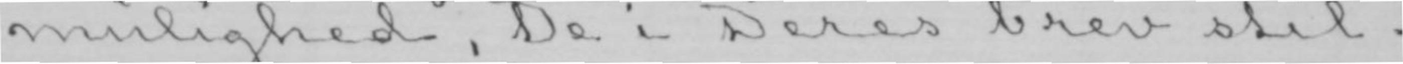mulighed, De i Deres brev stil-
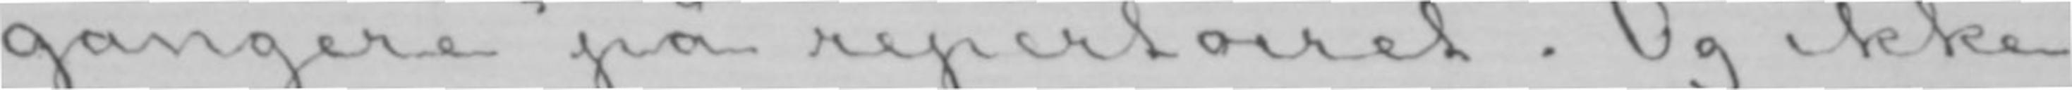gangere» på repertoiret. Og ikke
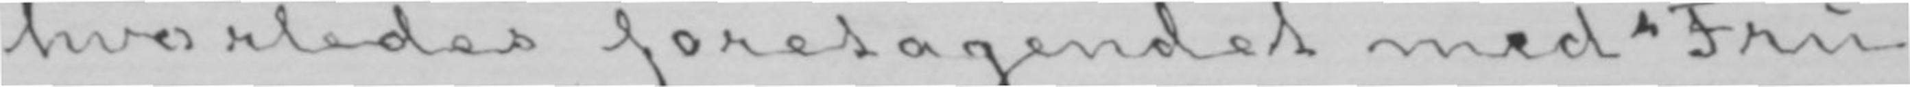hvorledes foretagendet med «Fru
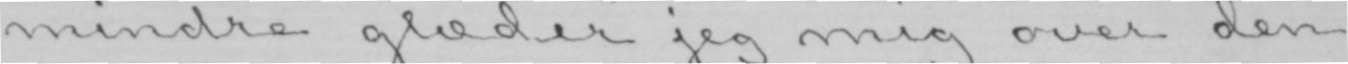mindre glæder jeg mig over den
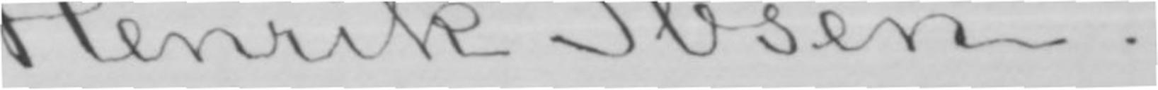Henrik Ibsen.
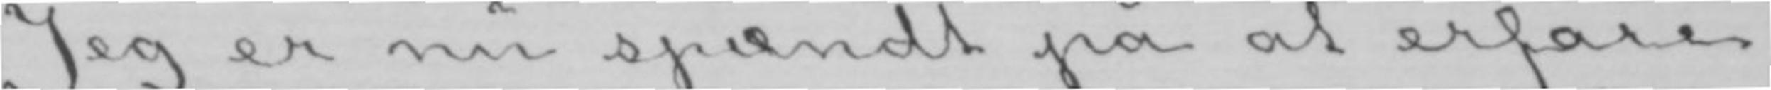Jeg er nu spændt på at erfare
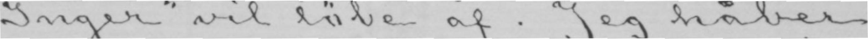Inger» vil løbe af. Jeg håber
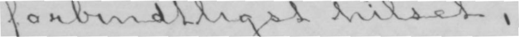forbindtligst hilset,
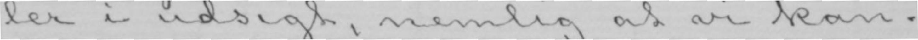ler i udsigt, nemlig at vi kan-
